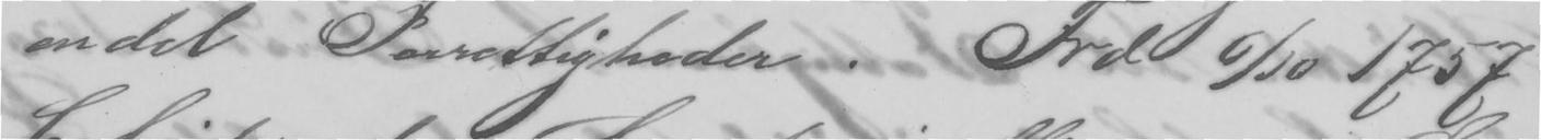endel Forrettigheder. Frd 6/10 1757
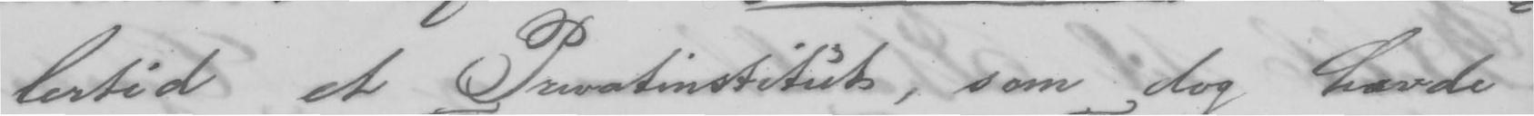lertid et Privatinstitut, som dog havde
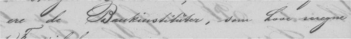ere de Bankinstituter, som have særegne
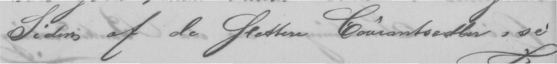Siden af de slettere Courantsedler, se'
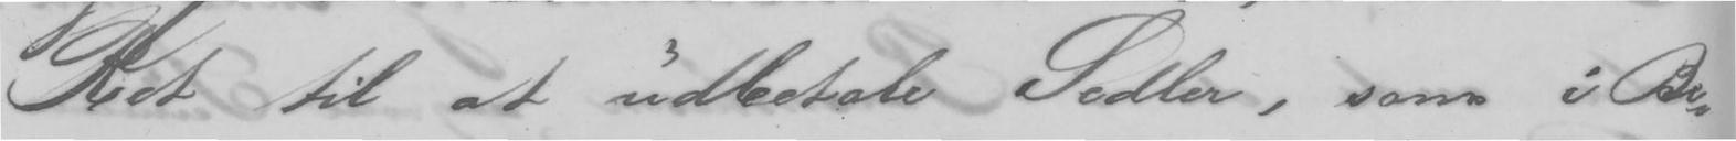Ret til at udbetale Sedler, som i Be-
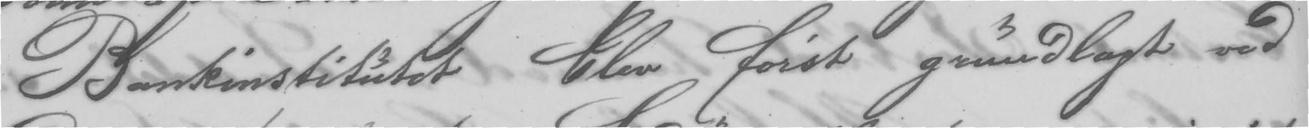Bankinstitutet blev først grundlagt ved
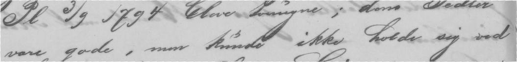vare gode, men kunde ikke holde sig ved
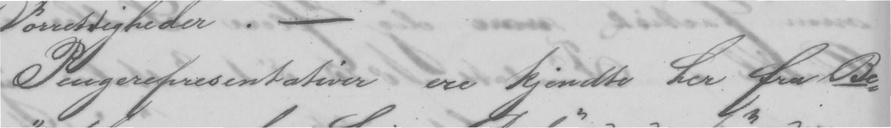Pengerepresentativer er kjendte her fra Be-
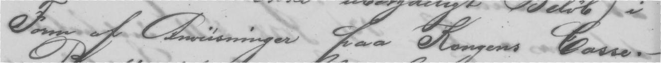Form af Anvisninger paa Kongens Casse. -
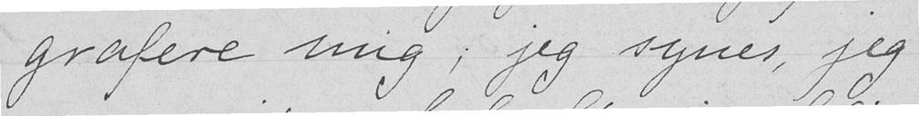grafere mig; jeg synes, jeg
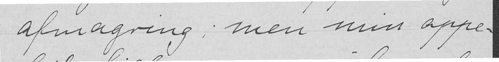afmagring, men min appe-
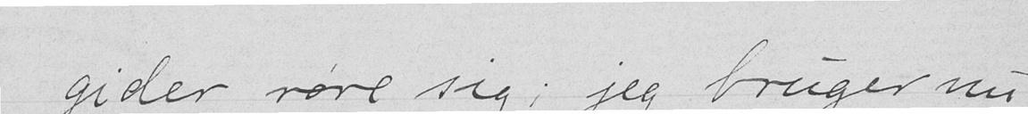gider røre sig; jeg bruger nu
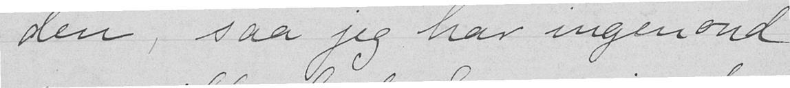den, saa jeg har ingen ond
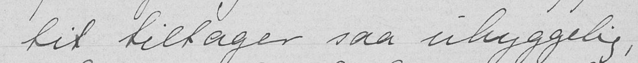tit tiltager saa uhyggelig,
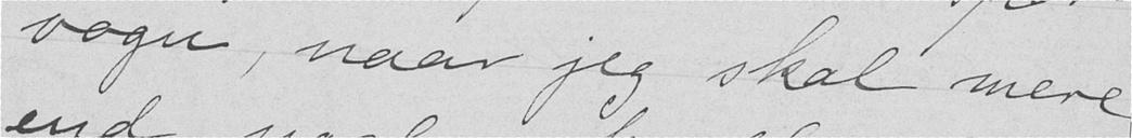vogn, naar jeg skal mere
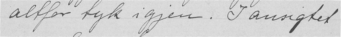altfor tyk igjen. I ansigtet
